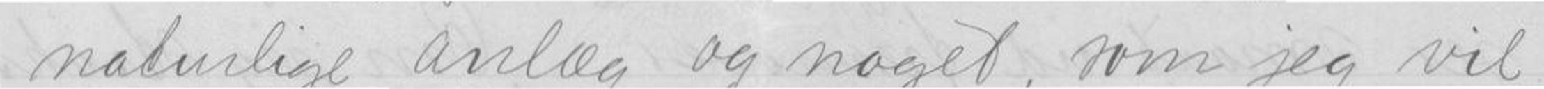naturlige anlæg og noget, som jeg vil
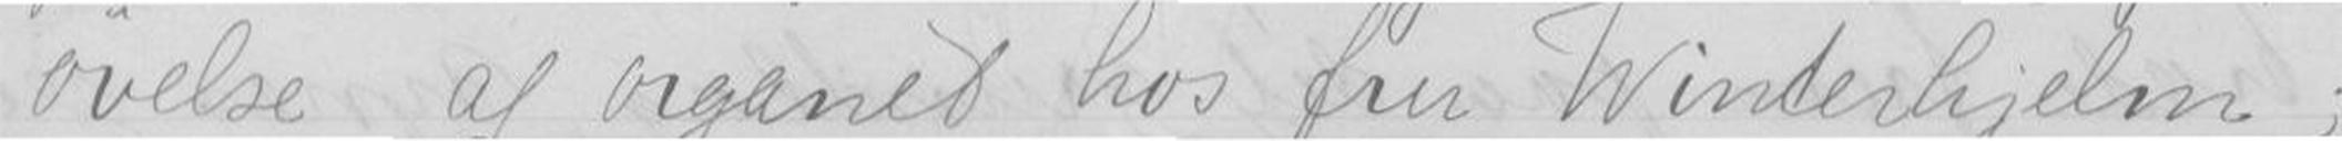øvelse af organet hos Winterhjelm;
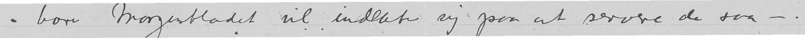– bare Morgenbladet vil, indlate sig paa at servere de saa –.
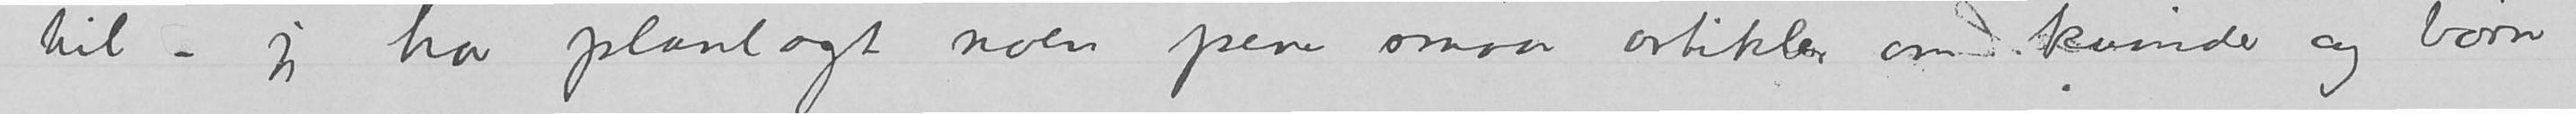til – jeg har planlagt noen pene smaa artikler om kvinder og børn
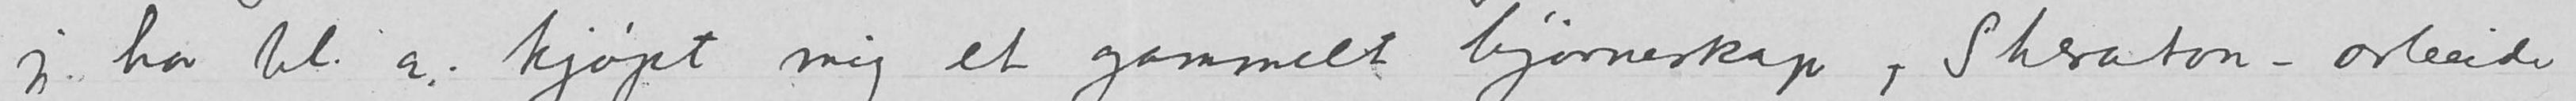jeg har bl. a. kjøpt mig et gammelt hjørneskap, Sheraton – arbeide
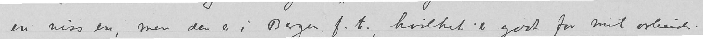en viss en, men den er i Bergen f.t. hvilket er godt for mit arbeide.
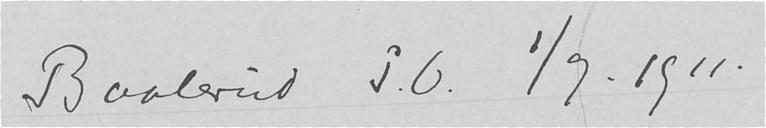Baalerud P. B. 1/9. 1911.
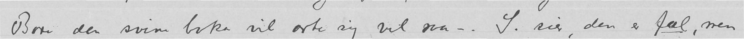Bare den svine boke vil arte sig vel saa –. S. sier, den er fæl, men
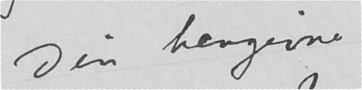Din hengivne
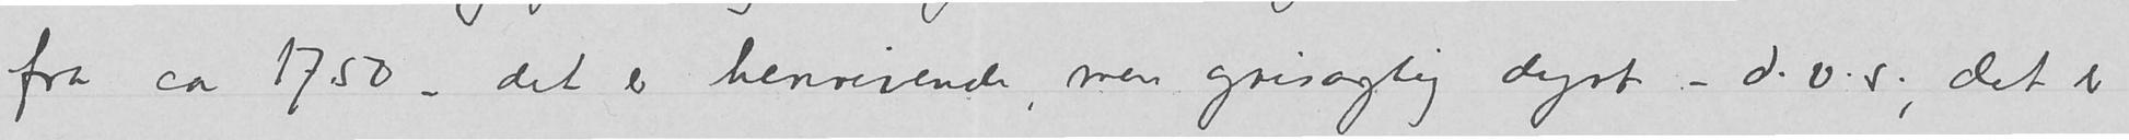fra ca 1750 – det er henrivende, men grisagtig dyrt – d.v.s., det er
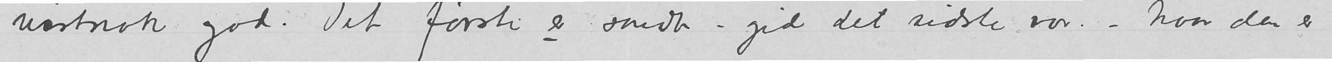vistnok god. Det første er sandt – gid det sidste var. – Naar den er
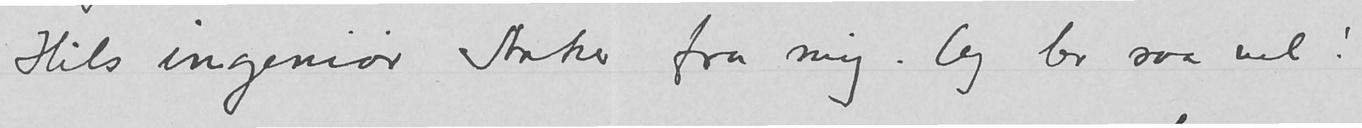Hils ingeniør Anker fra mig. Og lev saa vel!
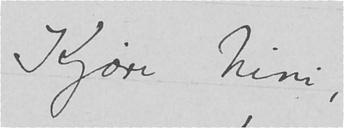Kjære Nini,
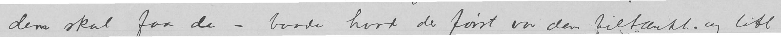dem skal faa de – baade hvad der først var dem, tiltænkt og litt
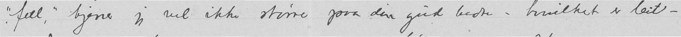«fæl,» tjener jeg vel ikke større paa din gud bedre – hvilket er leit –
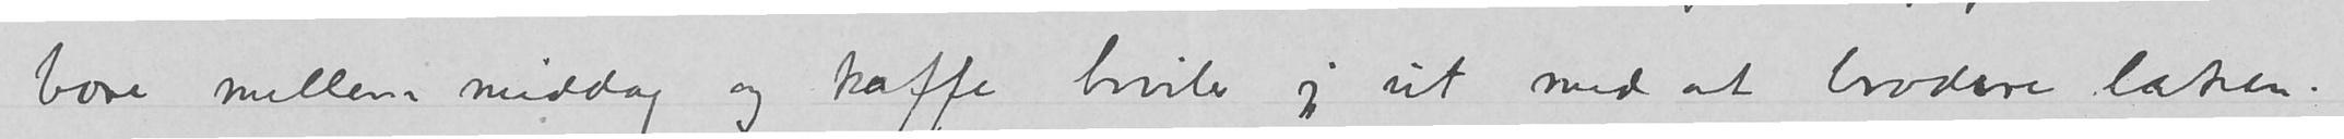bare mellem middag og kaffe hviler jeg ut med at brodere laken.
